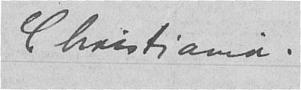Christiania.
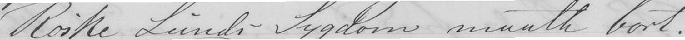Roske Lunds Sygdom maatte bort-
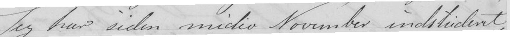Jeg har siden midio November indstuderet,
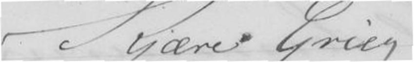Kjære Grieg!
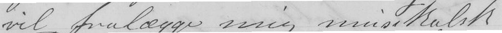vil fralægge mig musikalsk
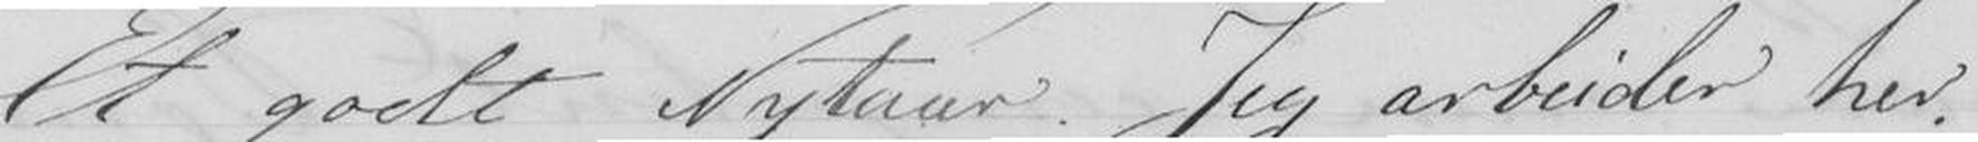Et godt Nytaar. Jeg arbeider her
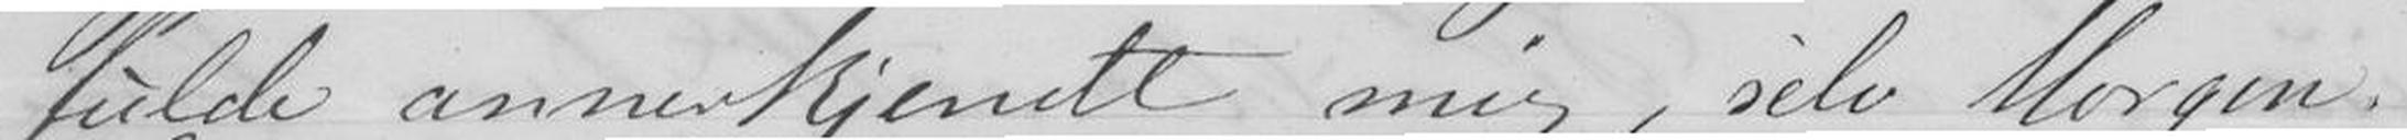fulde annerkjendt mig, selv Morgen-
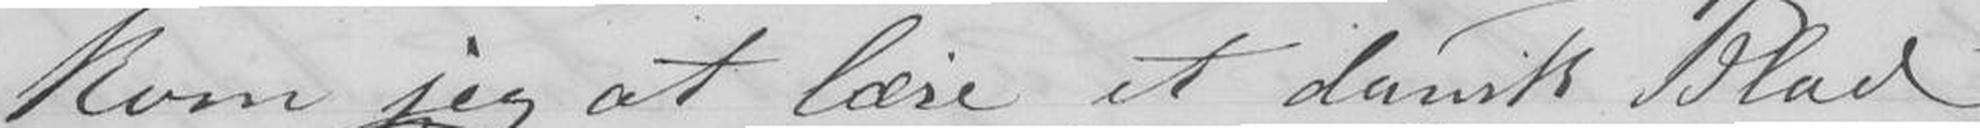kom jeg at læse et dansk Blad
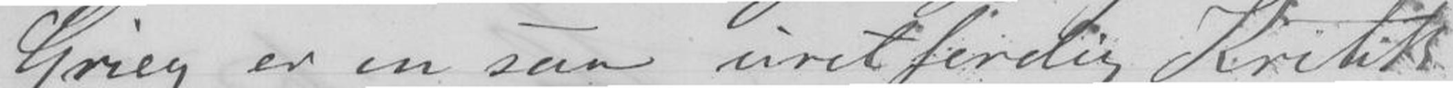Grieg er en saa uretferdig Kritik
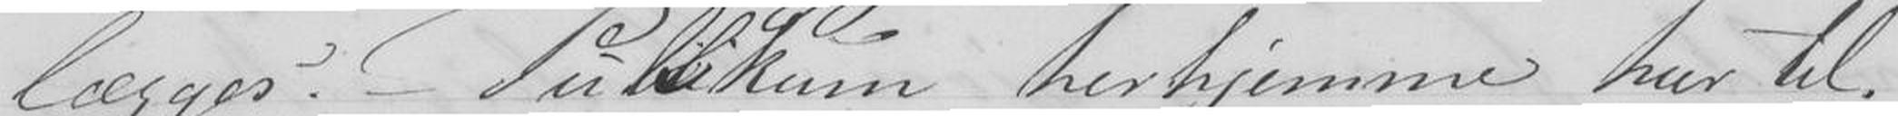lægges. - Publikum herhjemme har til-
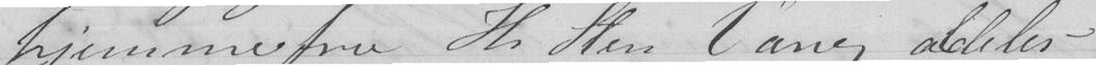hjemmefra Hr Sten Vang aldeles
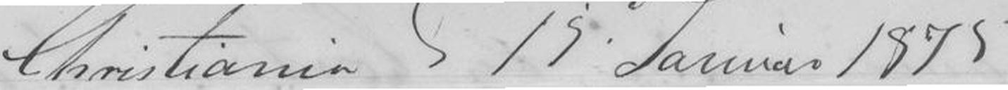Christiania d 19 Januar 1875
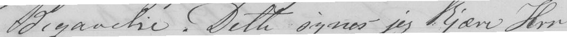Begavelse. Dette synes jeg Kjære Hrr
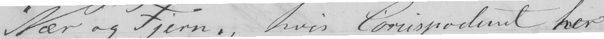Nær og Fjern", hvis Coruspodenet her
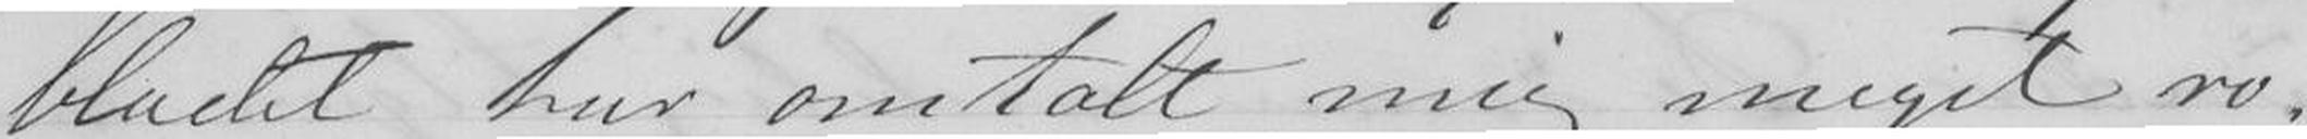bladet har omtalt mig meget ro
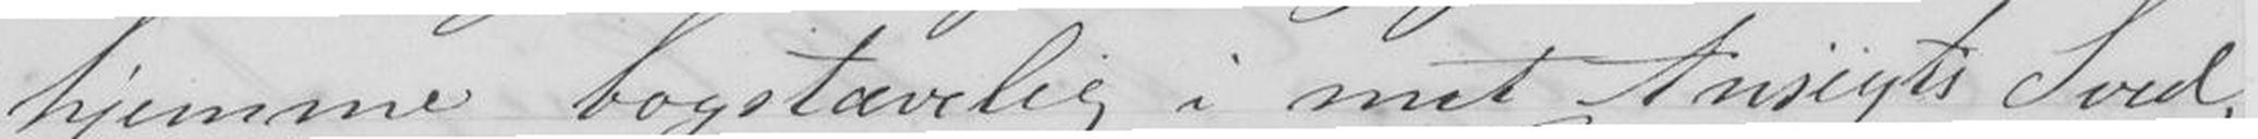hjemme bogstavelig i mit Ansigts Sved,
